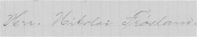Herr. Nikolai Frøsland.
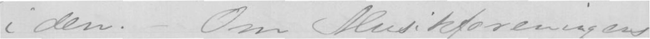i den. - Om Musikforeningens
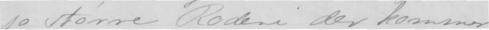jo større Roderi der kommer
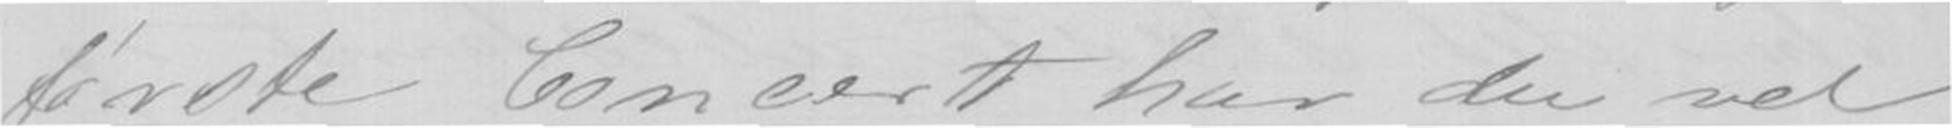første Concert har du vel
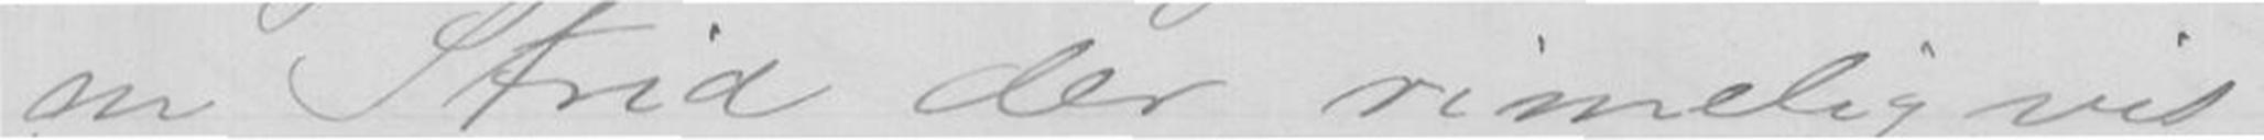om Strid der rimeligvis
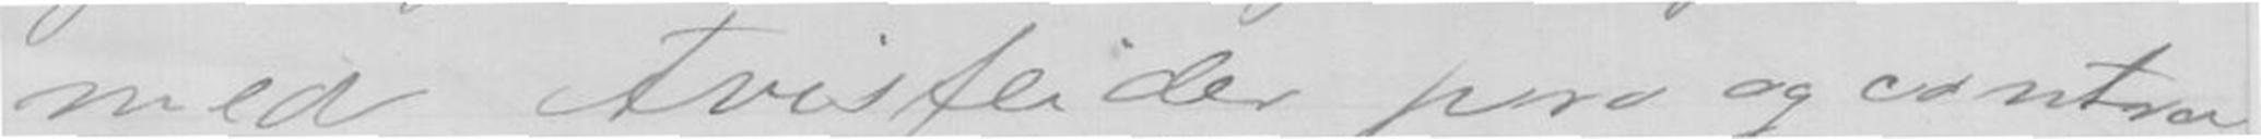med Avisfeider pro og contra
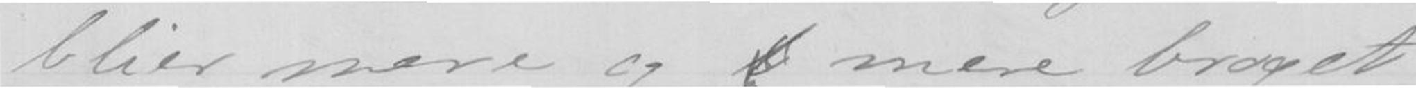blier mere og mere broget
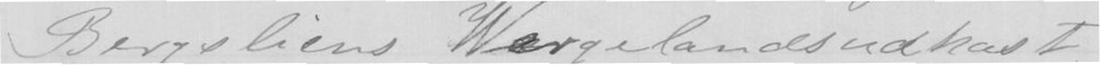Bergsliens Wergelands udkast,
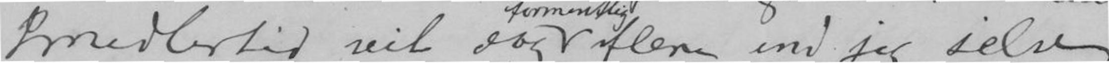Imidlertid vil dog flere end jeg selv
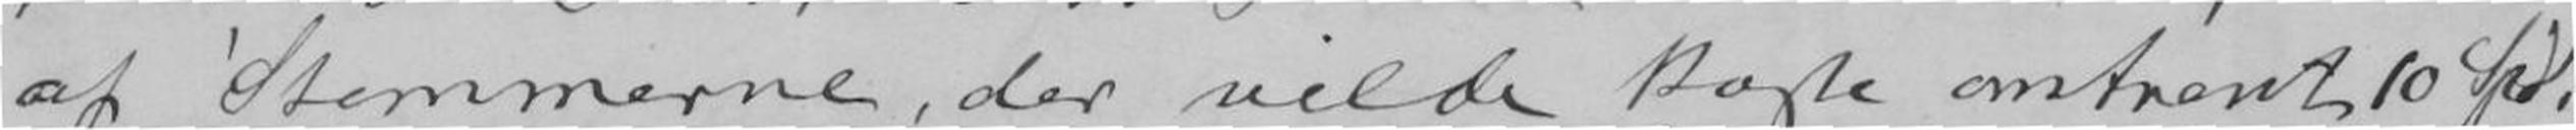af Stemmerne, der vilde koste omtrent 10 Spd.
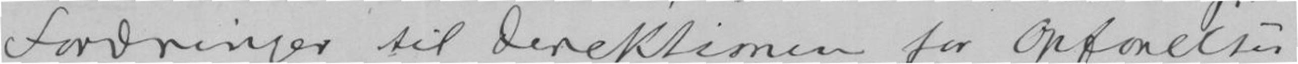fordringer til Direktionen for Opførelser
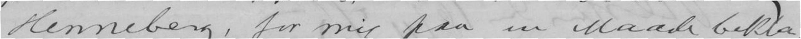Henneberg, for mig paa en Maade bekla-
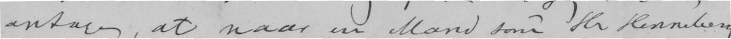antage, at naar en Mand som Hr Henneberg
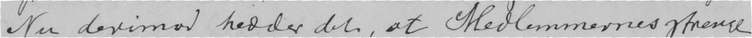Nu derimod hedder det, at Medlemmernes strenge
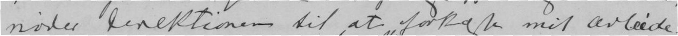nøder Direktionen til at forkaste mit arbeide.
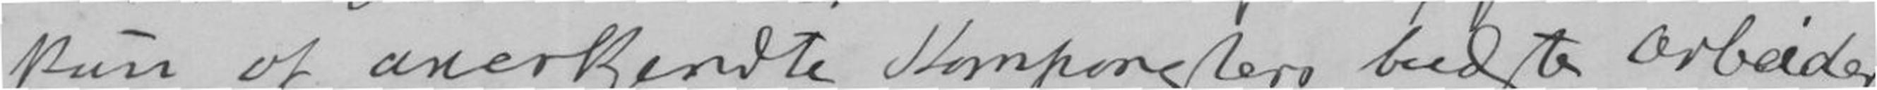kun af anerkjendte Komponisters bedste arbeide.
